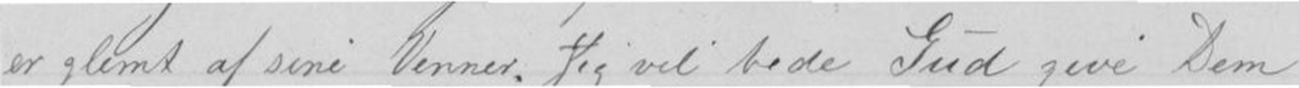er glemt af sine Venner. Jeg vil bede Gud give Dem
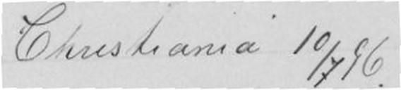Christiania 10/7 96.
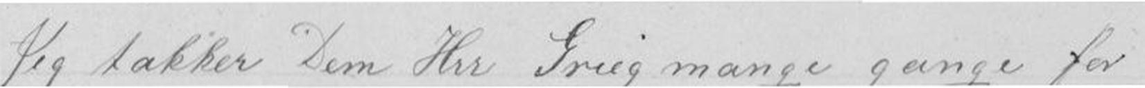Jeg takker Dem Hrr Grieg mange gange for
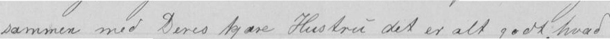sammen med Deres kjære hustru det er alt godt, hvad
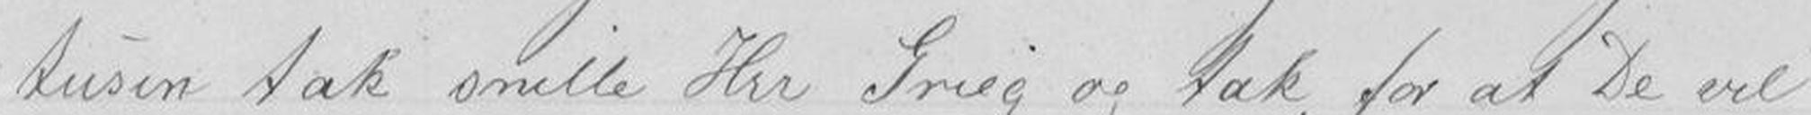tusen tak snille Hrr Grieg og tak for at De vil
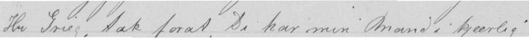Hrr Grieg, tak forat De har min Mand i kjærlig
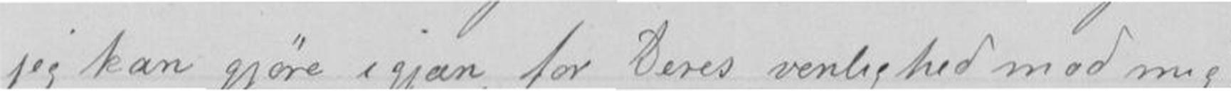jeg kan gjøre igjæn, for Deres venlighed mod mig,
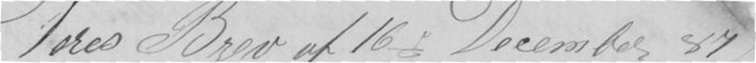Deres Brev af 16de December 87
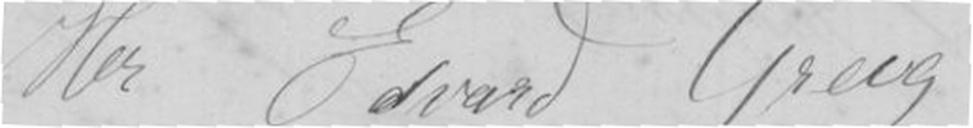Herr Edvard Grieg
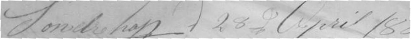Sondre hop d. 28de April 188
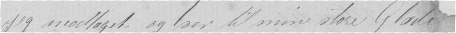jeg modtaget og ser til min store Glæde
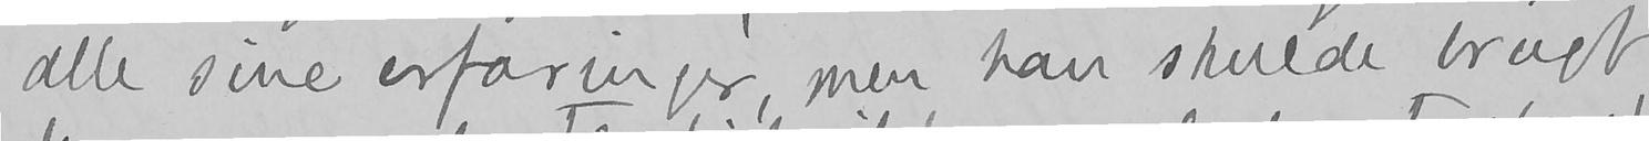alle sine erfaringer, men han skulde brugt
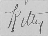Kitty
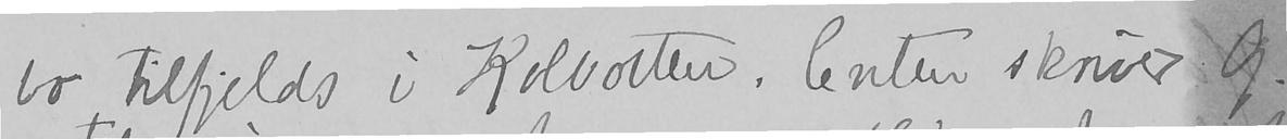bo tilfjelds i Kolbotten. Enten skriver G.
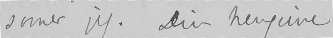sovner jeg. Din hengivne
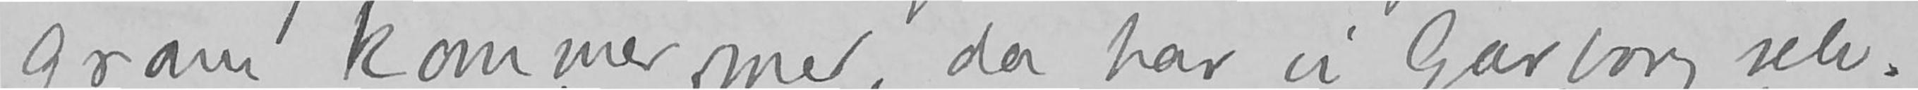Gram kommer med, da har vi Garborg selv.
